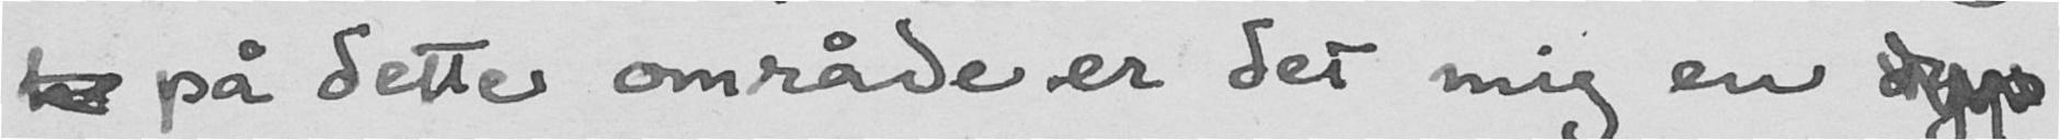h på dette område er det mig en dyp
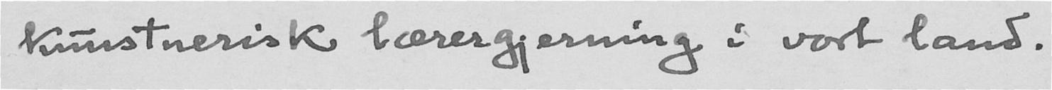kunstnerisk lærergjerning i vort land.
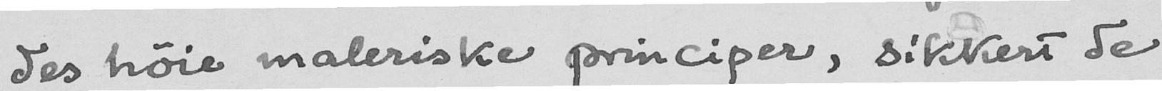des høie maleriske principer, sikkert de
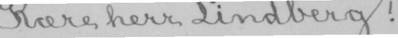Kære herr Lindberg!
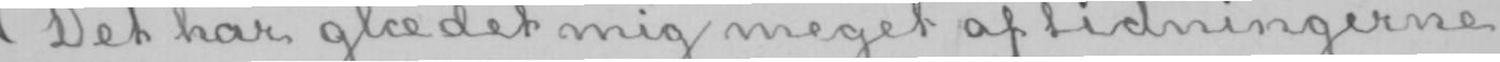Det har glædet mig meget af tidningerne
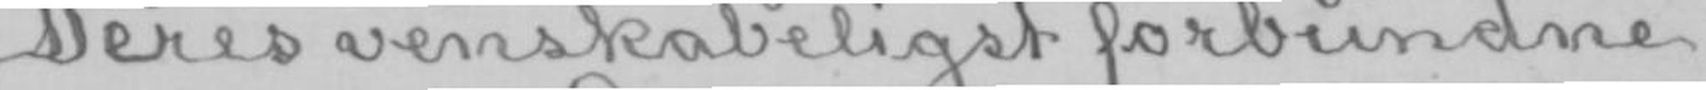Deres venskabeligst forbundne
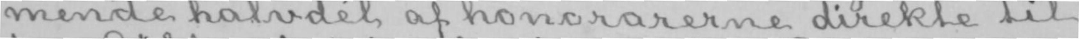mende halvdél af honorarerne direkte til
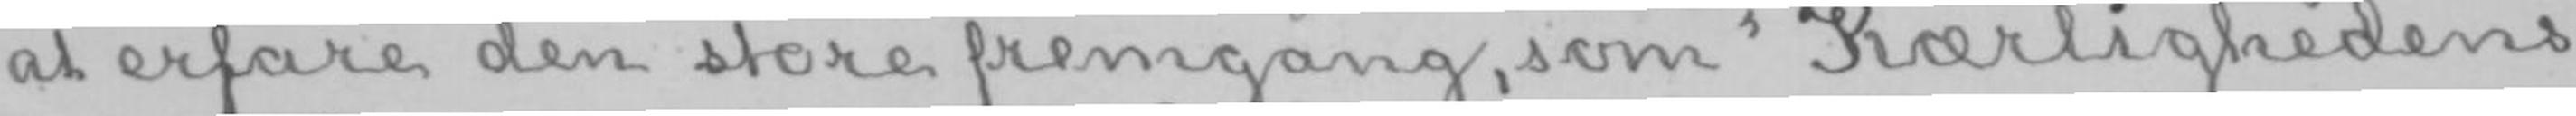at erfare den store fremgang, som «Kærlighedens
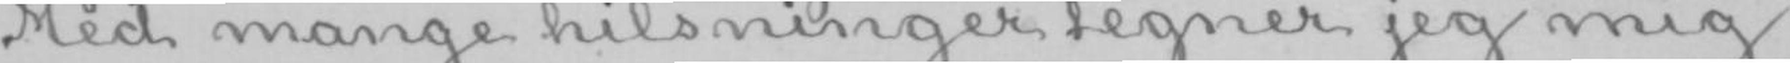Med mange hilsninger tegner jeg mig
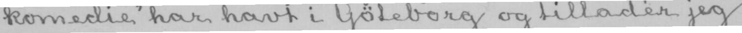komedie» har havt i Göteborg og tillader jeg
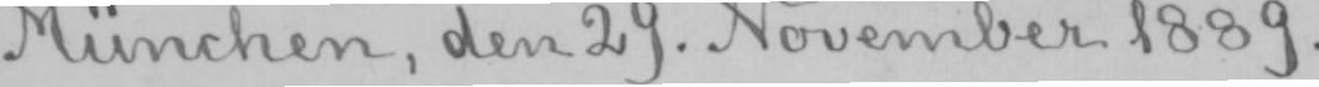München, den 29. November 1889.
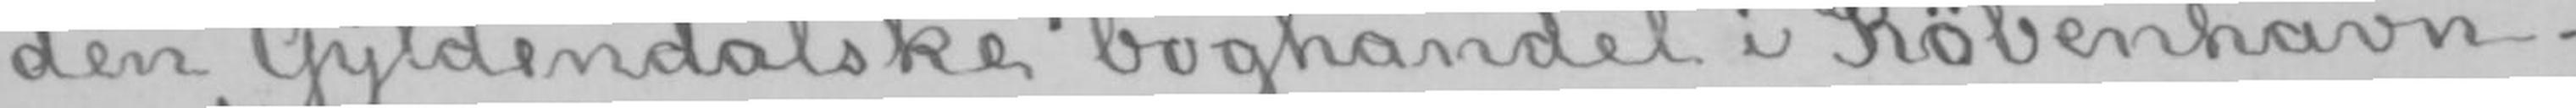den Gyldendalske boghandel i København.
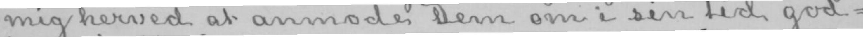mig herved at anmode Dem om i sin tid god-
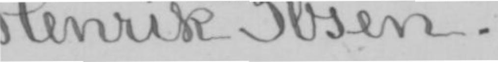Henrik Ibsen.
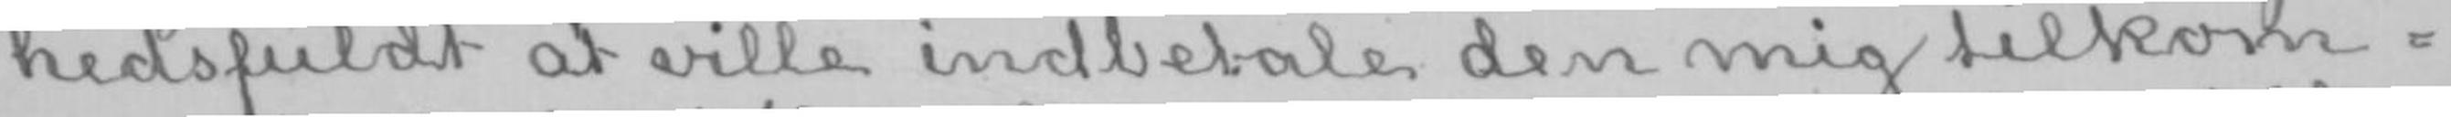hedsfuldt at ville indbetale den mig tilkom-
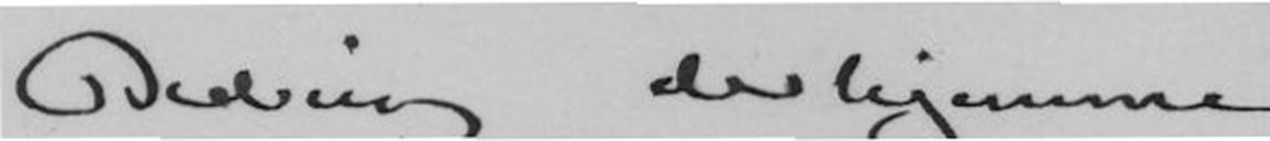Bedring derhjemme
